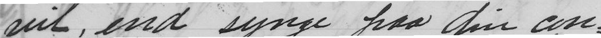vil, end synge paa din con-
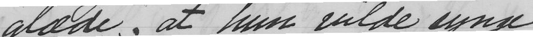glæde, at hun vilde synge
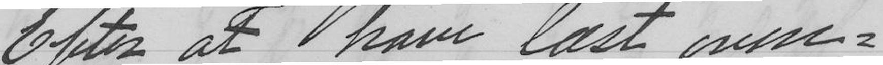Efter at have læst oven-
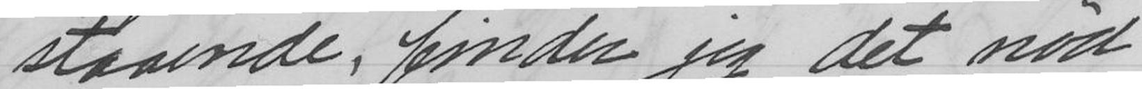staaende, finder jeg det nød-
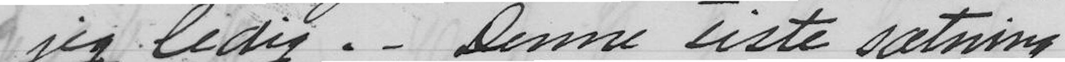jeg ledig. - Denne siste sætning
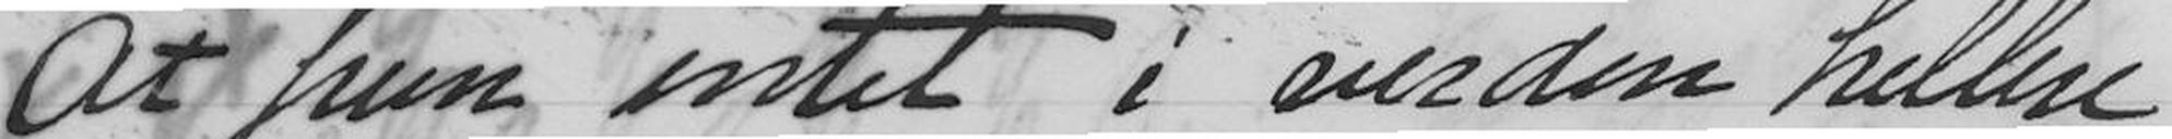At hun intet i verden hellere
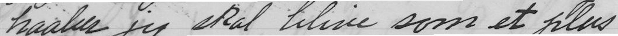haaber jeg skal blive som et plus
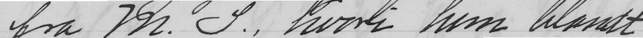fra M. S., hvori hun blandt
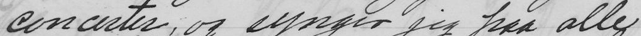concerter, og synger jeg paa alle
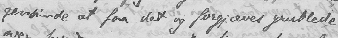gensinde at faa det og forgjæves grublede
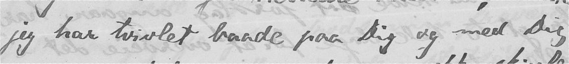jeg har tvivlet baade paa Dig og med Dig
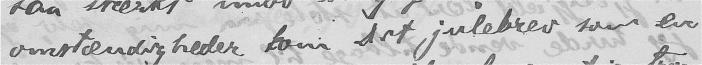omstændigheder kom Dit julebrev som en
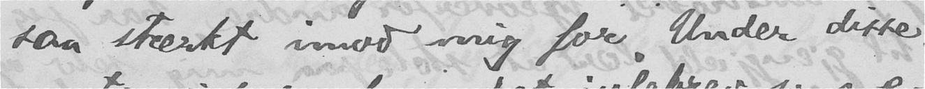saa stærkt imod mig for. Under disse
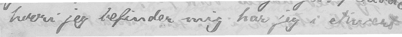hvori jeg befinder mig har jeg i ethvert
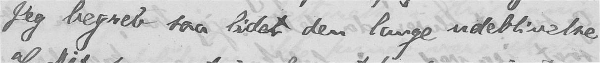Jeg begreb saa lidet den lange udeblivelse
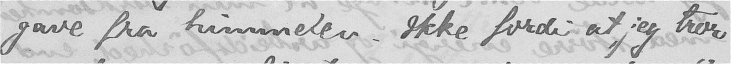gave fra himmelen. Ikke fordi at jeg tror
